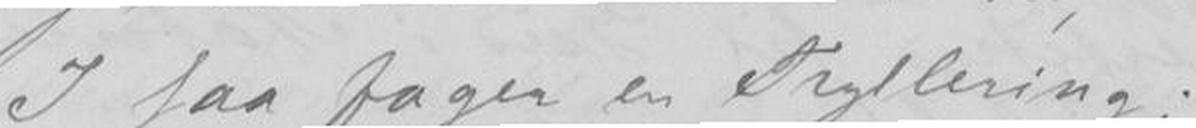I saa fager en Tryllering;
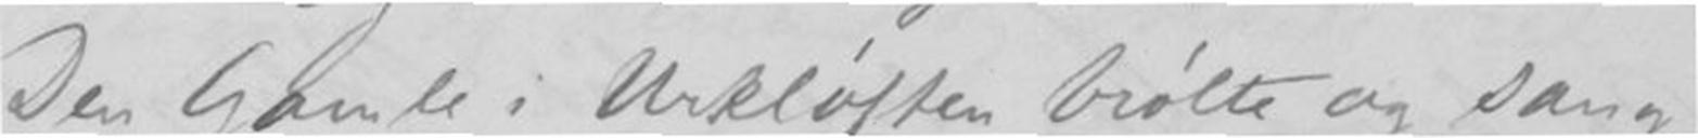Den Gamle i Urkløften brølte og sang
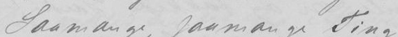Saamange, saamange Ting,
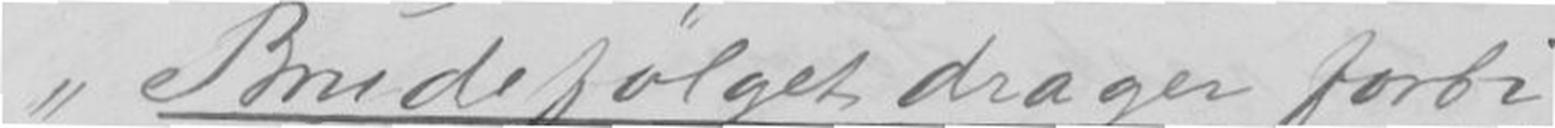Brudefølget drager forbi
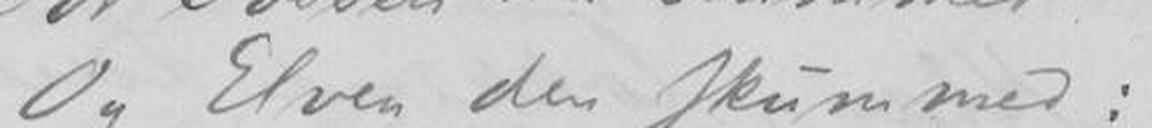Og Elven den skummed:
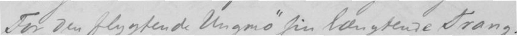For den flygtende Ungmø sin længende Trang;
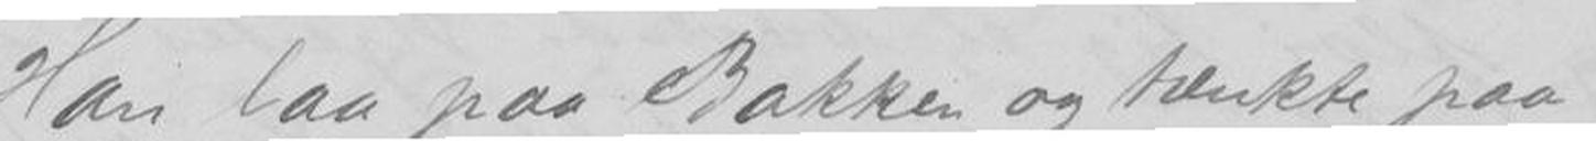Han laa paa Bakken og tænkte paa
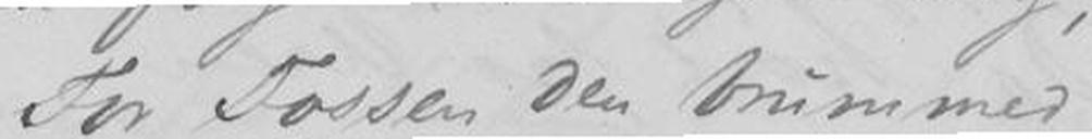For Fossen den brummed
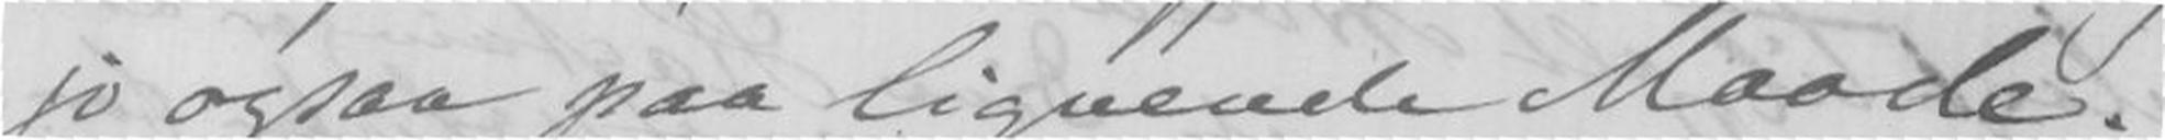jo ogsaa paa lignende Maade.
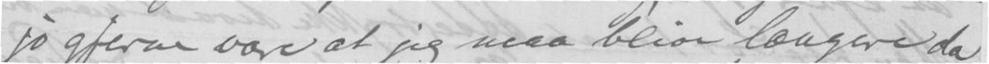jo gjerne være at jeg maa blive længere da
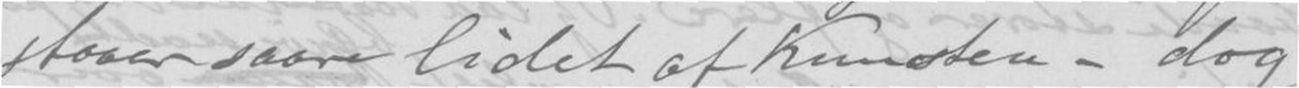staar saare lidet af Kunsten - dog
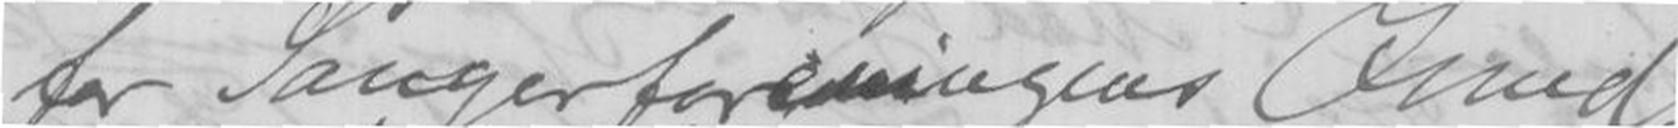for Sangerforeningens Fond.
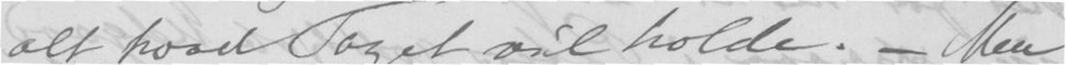alt hvad Toget vil holde. - Men
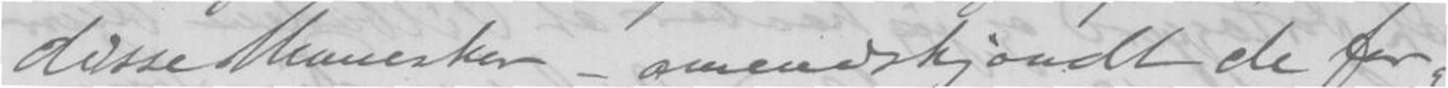disse Mennesker - omendskjøndt de for-
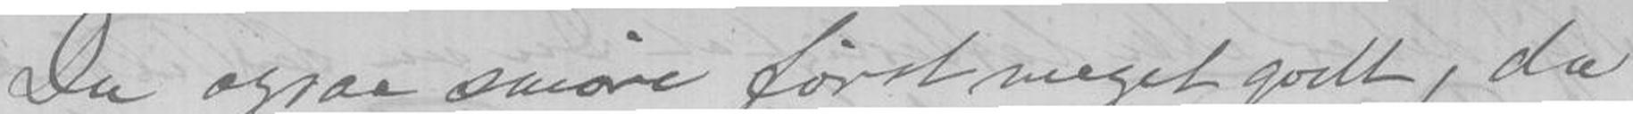Du ogsaa smøre først meget godt, da
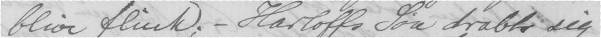blive flink; - Harloffs Søn drabte sig
